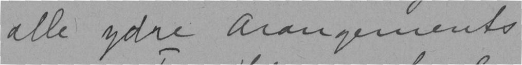alle ydre Arangements
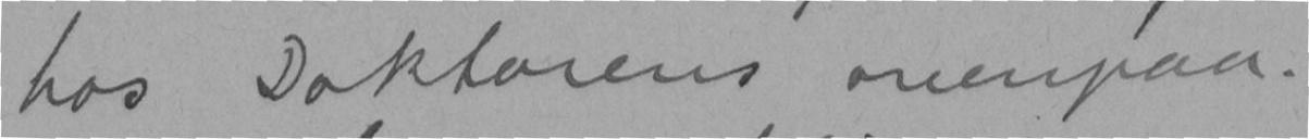hos Doktorens ovenpaa.
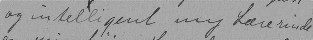og intelligent ung Lærerinde
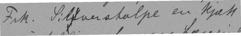Frk. Silverstolpe en kjæk
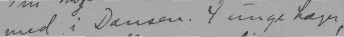med i Dansen. 4 unge læger,
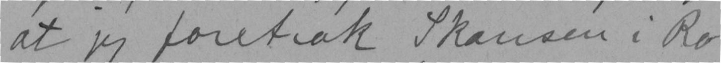at jeg foretrak Skansen i Ro
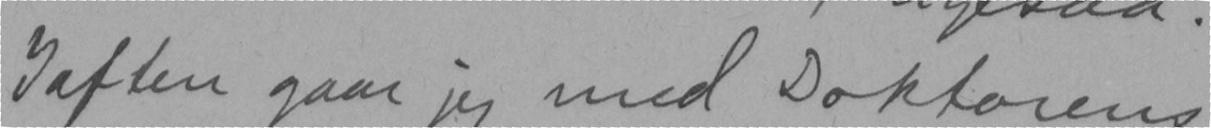Iaften gaar jeg med Doktorens
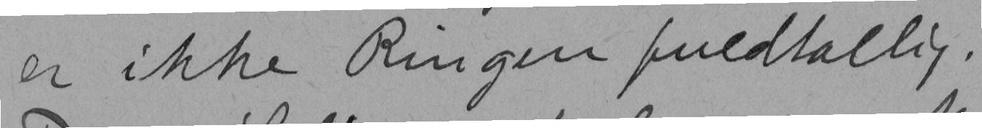er ikke Ringen fuldtallig.
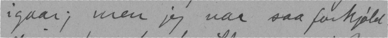igaar; men jeg var saa forkjøld
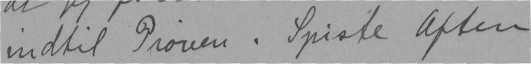indtil Prøven. Spiste aften
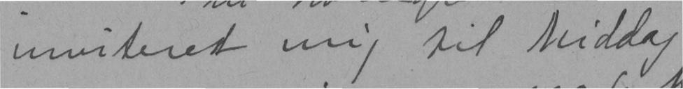inviteret mig til Middag
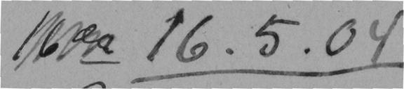16.5.04
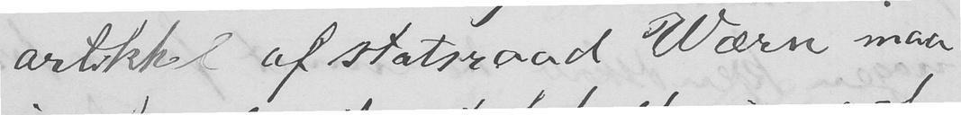artikkel af statsraad Wærn maa
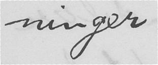ninger.
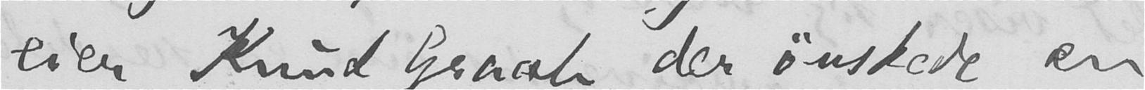eier, Knud Graab, der ønskede en
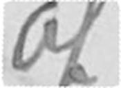af
